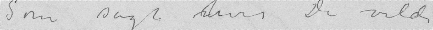Som sagt hvis De vilde
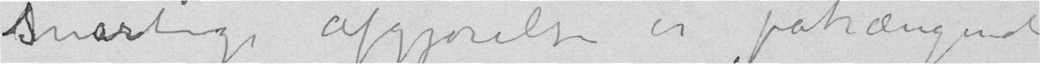snarlige Afgjørelse er påtrængende
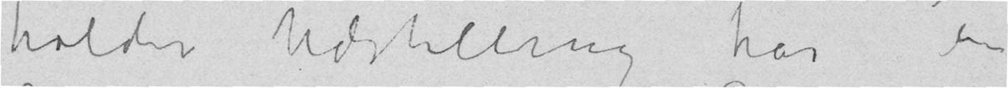holder Udstilling har en
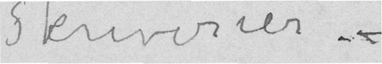Skriverier. –
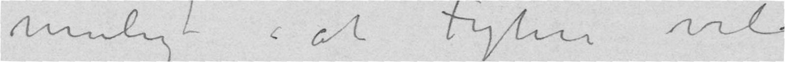muligt at Fyhn vil
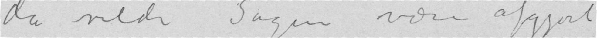da vilde Sagen være afgjort
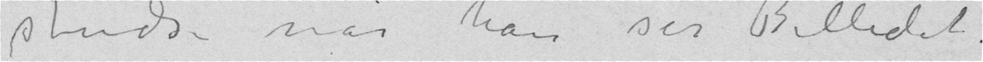studse når han ser Billedet –
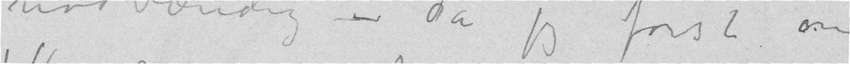nødvændig – da jeg først om
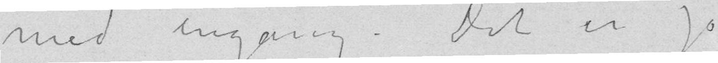med engang. Det er jo
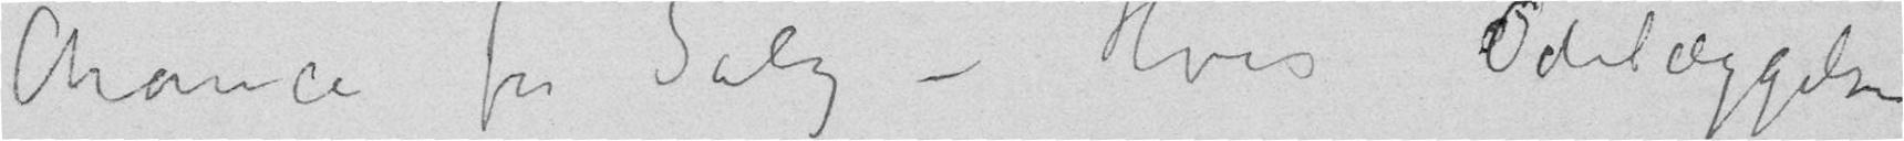Chance for Salg – Hvis Ødelæggelse
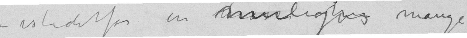– istedetfor muligvisens mange
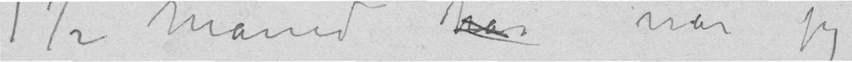l ½ Måned har når jeg
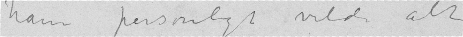ham personligt vilde alt
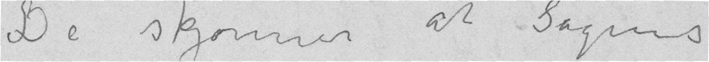De skjønner at Sagens
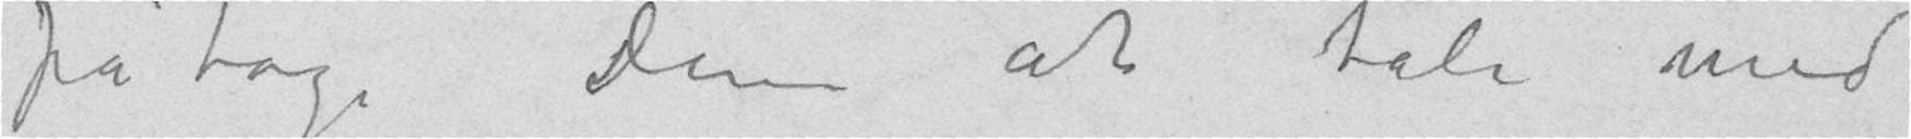påtage Dem at tale med
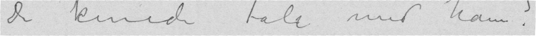De kunde tale med ham?
